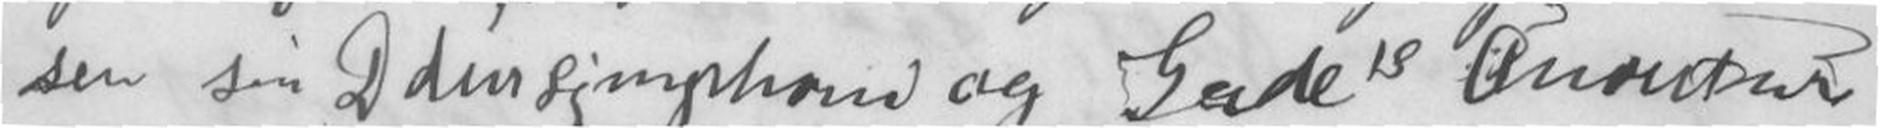sen sin D dursymphoni og Gade's Ouverture
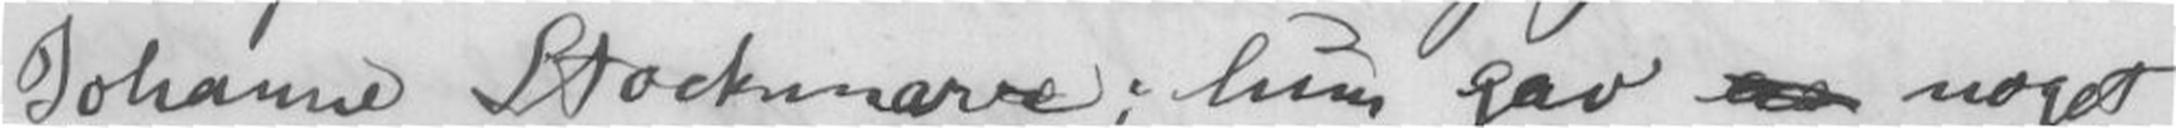Johanne Stockmarr; hun gav noget
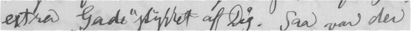extra Gade stykket af Dig. Saa var der
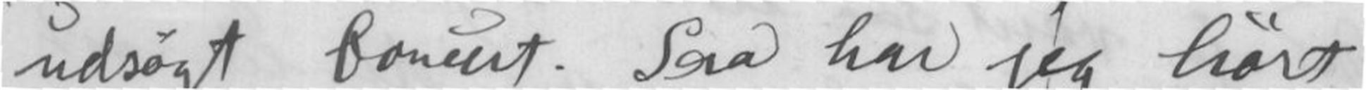udsøgt Concert. Saa har jeg hørt
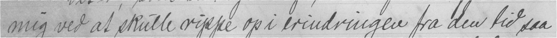mig ved at skulle rippe op i erindringen fra den tid saa
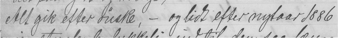Alt gik efter ønske, - og lidt efter nytaar 1886
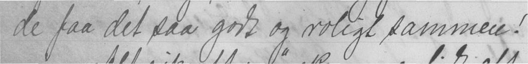de faa det saa godt og roligt sammen!
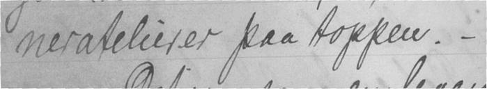neratelierer paa toppen. -
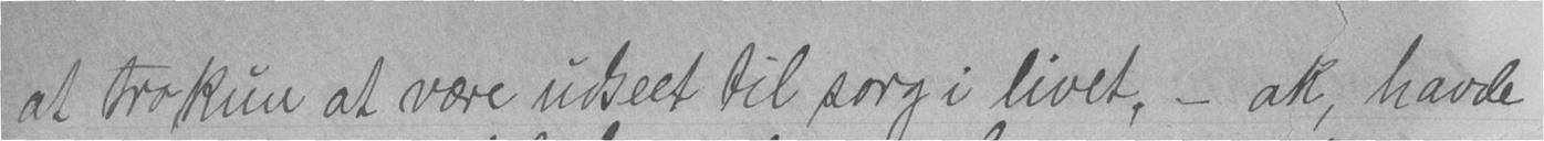at tro kun at være udseet til sorg i livet, - ak, havde
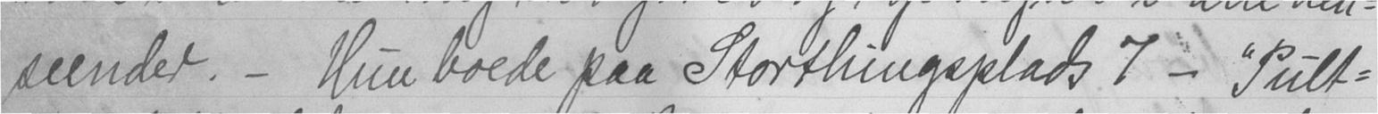seender. - Hun boede paa Storthingsplads 7 - "Pult-
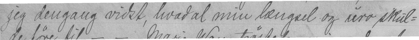jeg dengang vidst, hvad al min længsel og uro skul-
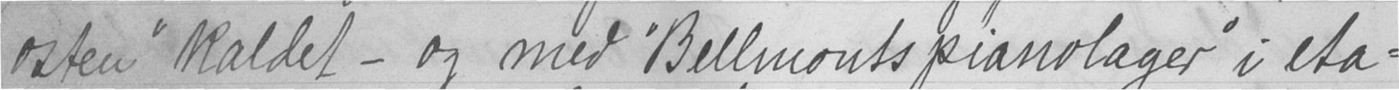osten" kaldet- og med "Bellmonts pianolager" i eta-
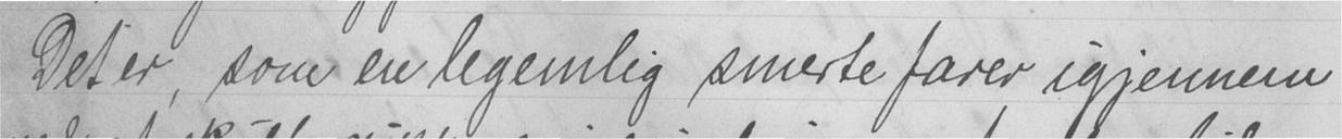Det er, som en legemlig smerte farer igjennem
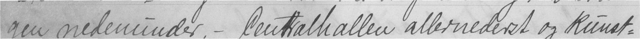gen nedenunder, - Centralhallen allernederst og kunst-
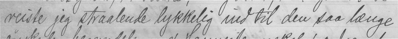reiste jeg straalende lykkelig ind til den saa lange
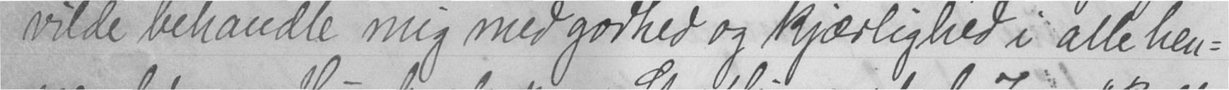vilde behandle mig med godhed og kjærlighed i alle hen-
